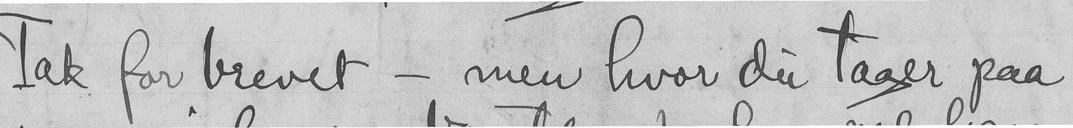Tak for brevet - men hvor du tager paa
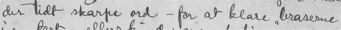der tidt skarpe ord - for at klare "braserne"
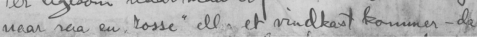naar saa en "rosse" eller et vindkast kommer - da
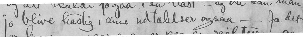jo blive hastig i sine udtalelser ogsaa - Ja det
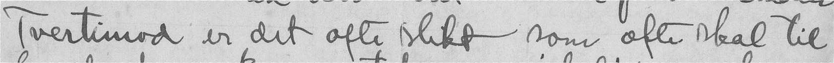Tvertimod er det ofte slikt som ofte skal til
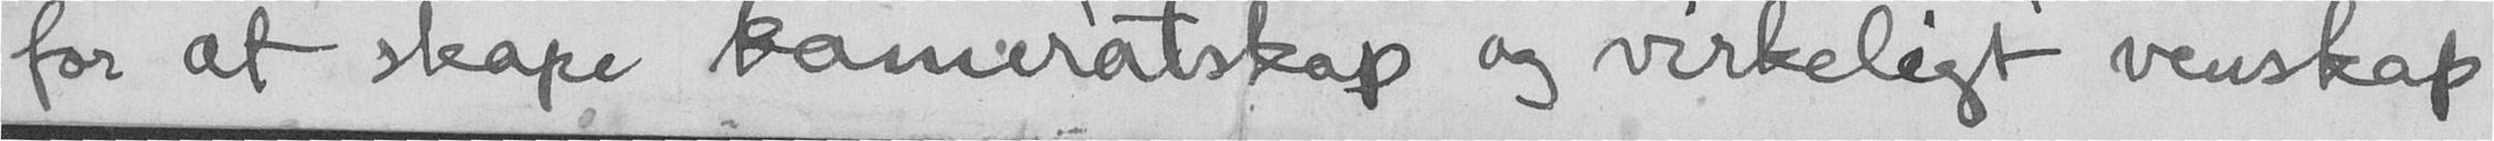for at skape kameratskap og virkeligt venskap
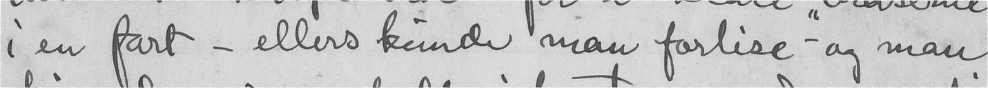i en fart - ellers kunde man forlise - og man
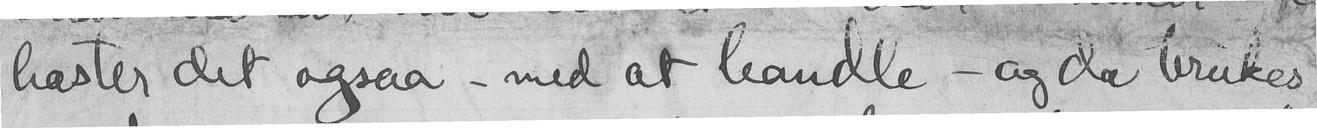haster det ogsaa - med at handle - og da brukes
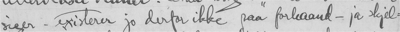siger - existerer jo derfor ikke paa forhaand - ja skjel-
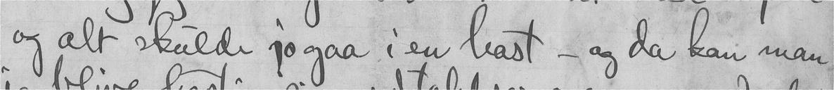og alt skulde jo gaa i en hast - og da kan man
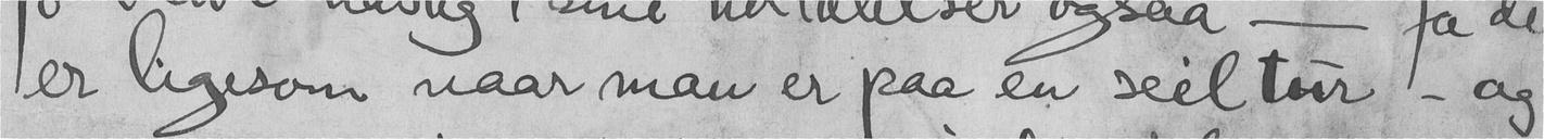er ligesom naar man er paa en seiltur - og
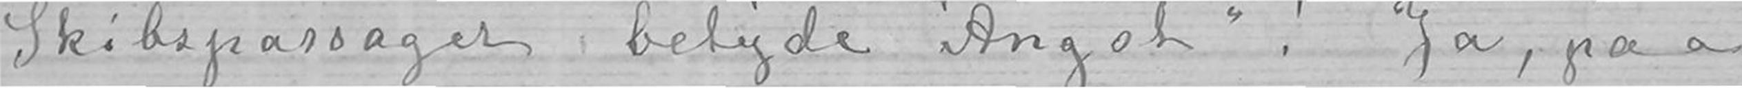Skibspassager betyde «Angst»! Ja, paa
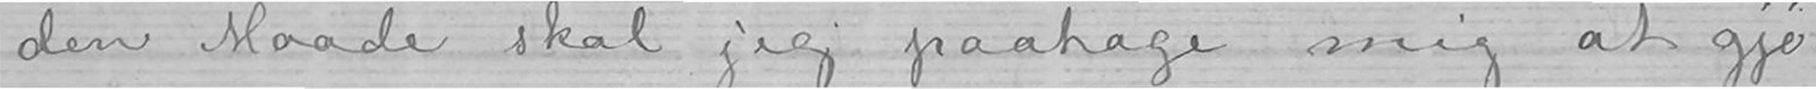den Maade skal jeg paatage mig at gjø-
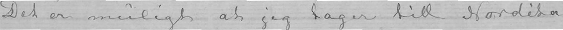Det er muligt at jeg tager till Nordita-
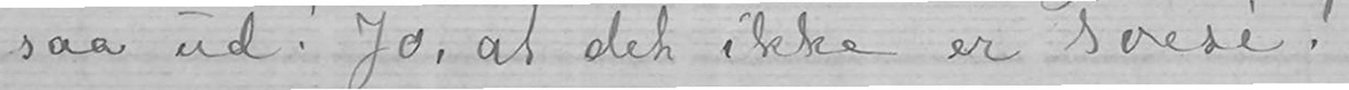saa ud! Jo, at det ikke er Poesi!-
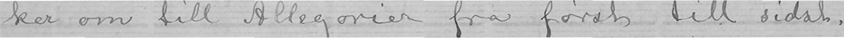ker om till Allegorier fra først till sidst.
